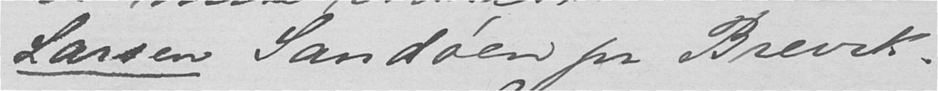Larsen Sandøen pr Brevik.
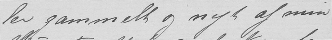ler gammelt og nyt af min
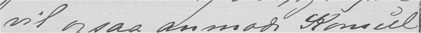vil ogsaa anmode Konsul
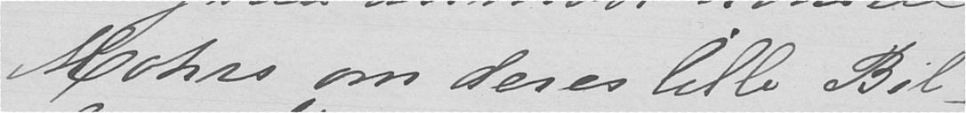Mohrs om deres lille Bil-
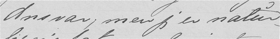Ansvar; men jeg er natur-
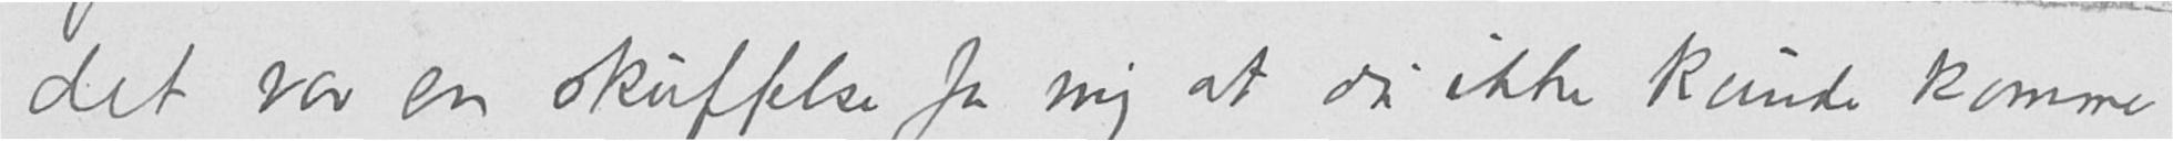det var en skuffelse for mig at du ikke kunde komme
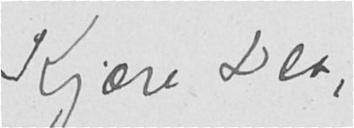Kjære Dea,
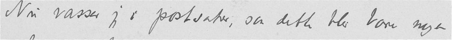Nu vasser jeg i postsaker, saa dette blir bare nogen
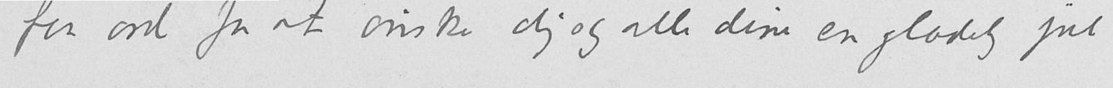faa ord for at ønske dig og alle dine en glædelig jul -
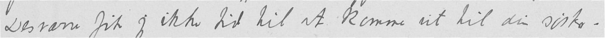Desværre fik jeg ikke tid til at komme ut til din søster -
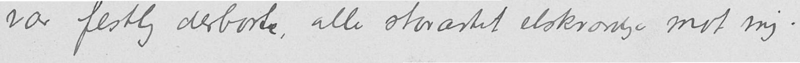var festlig derborte, alle storartet elskværdige mot mig.
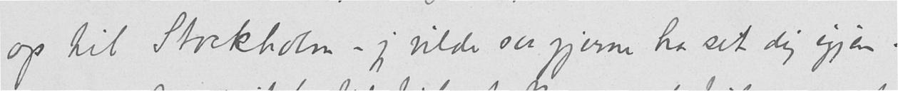op til Stockholm - jeg vilde saa gjerne ha set dig igjen.
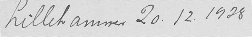Lillehammer 20.12.1928
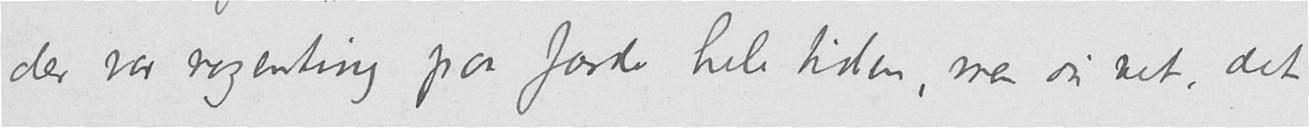der var nogenting paa færde hele tiden, som du vet, det
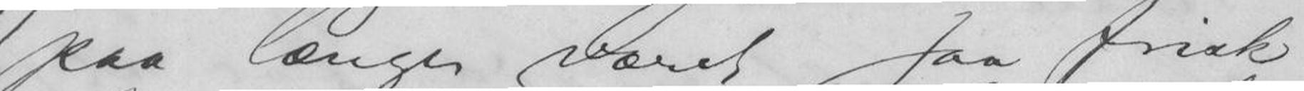paa længe været saa frisk
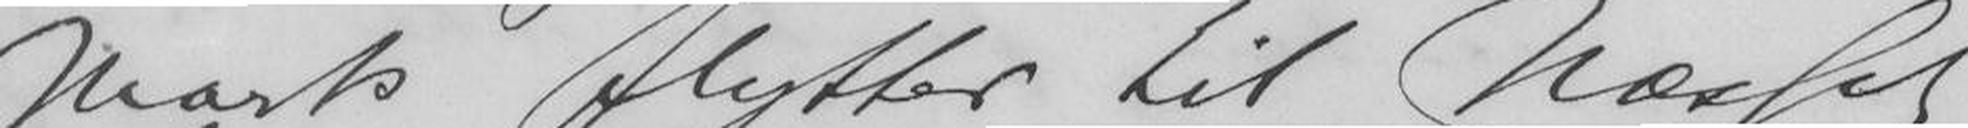Marts flytter til Næsset,
